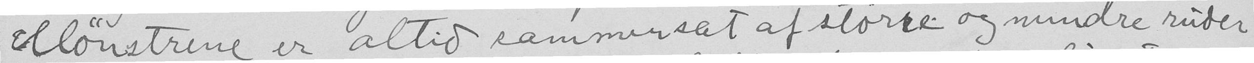Mønstrene er altid sammensat af større og mindre ruder
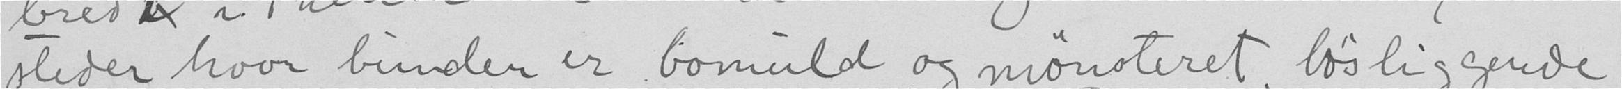steder hvor bunden er bomuld og mønsteret løsliggende
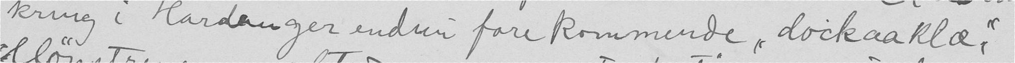kring i Hardanger endnu forekommende "dockaaklæ"
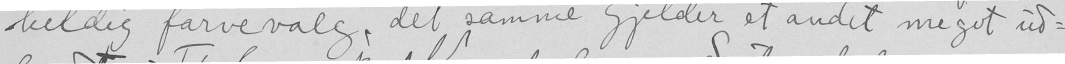heldig farvevalg, det samme gjelder et andet meget ud-
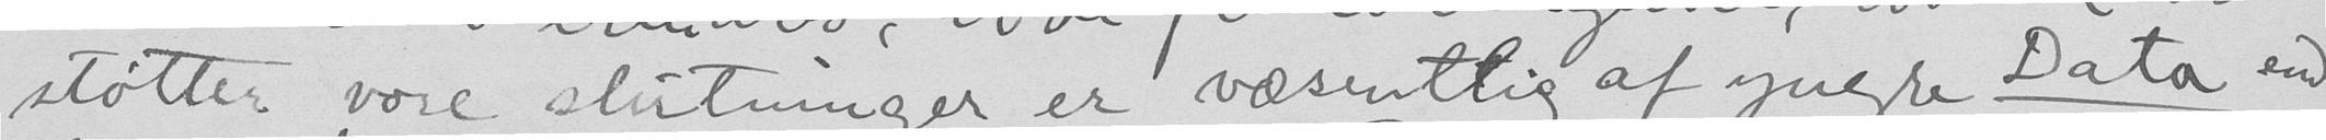støtter vore slutninger er væsentlig af yngre Data end
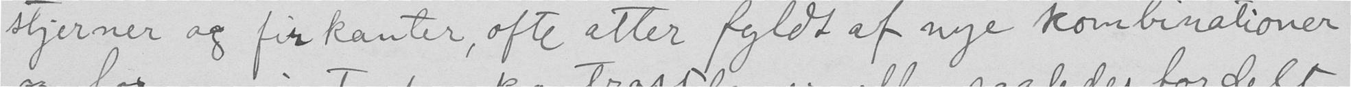stjerner og firkanter, ofte atter fyldt af nye kombinationer
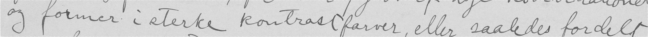og former i sterke kontrastfarver, eller saaledes fordelt
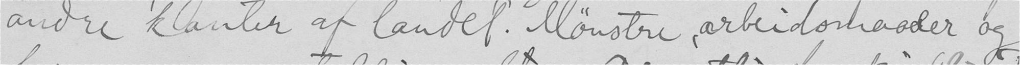andre kanter af landet. Mønstre, arbedsmaader og
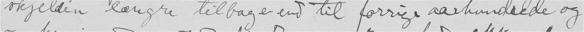skjelden længre tilbage end til forrige aarhundrede og
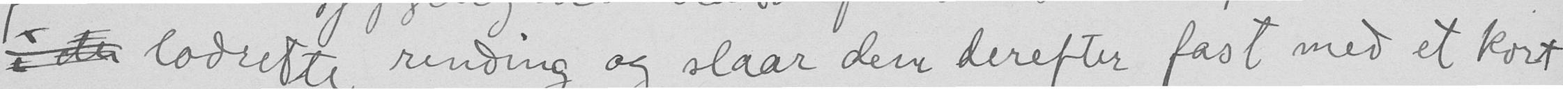lodrette rending og slaar den derefter fast med et kort
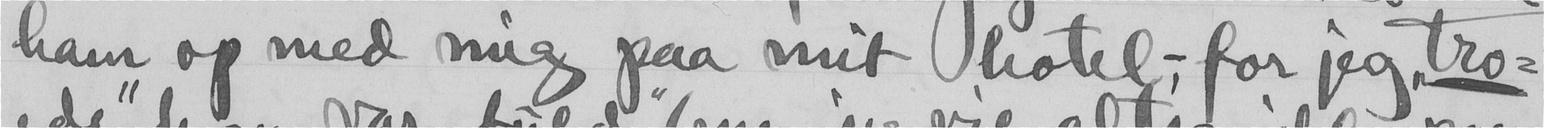ham op med mig paa mit hotel, for jeg "tro-
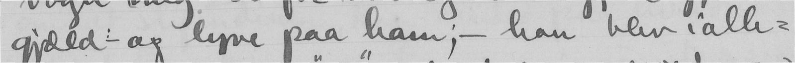gjæld - og lyve paa ham; - han blev ialle-
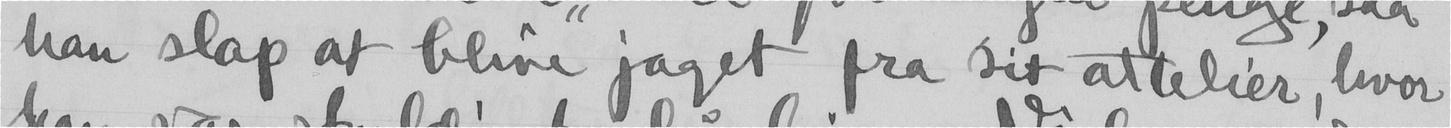han slap at blive jaget fra sit attelier, hvor
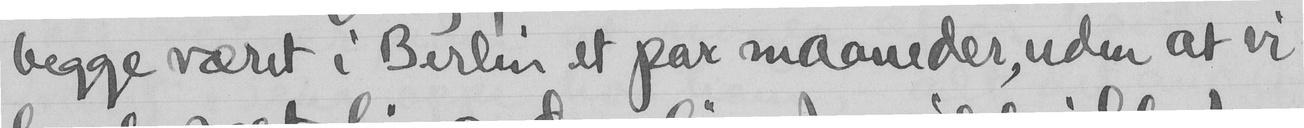begge været i Berlin et par maaneder, uden at vi
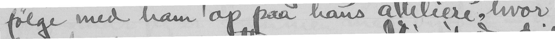følge med ham op paa hans atteliere, hvor
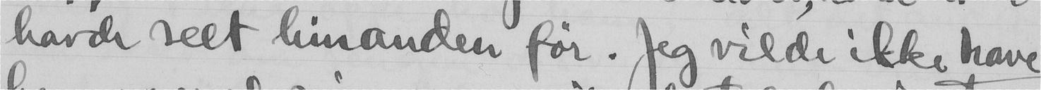havde seet hinanden før. Jeg vilde ikke have
